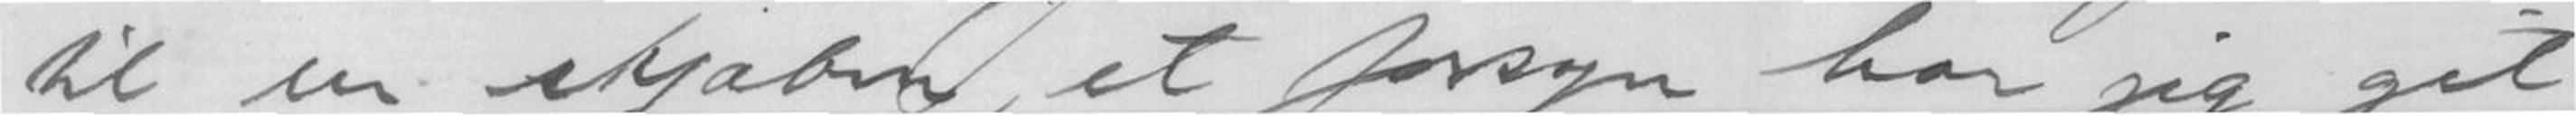til en skjæbne, et forsyn har jeg git
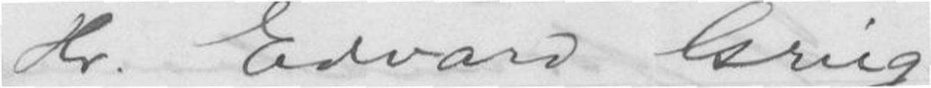Hr. Edvard Grieg
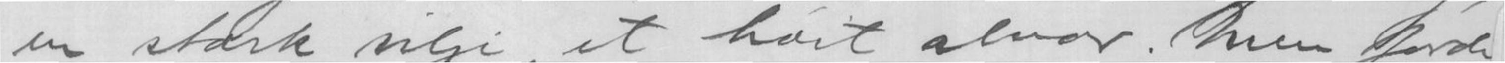en stærk vilje, et høit alvor. Kun fordi
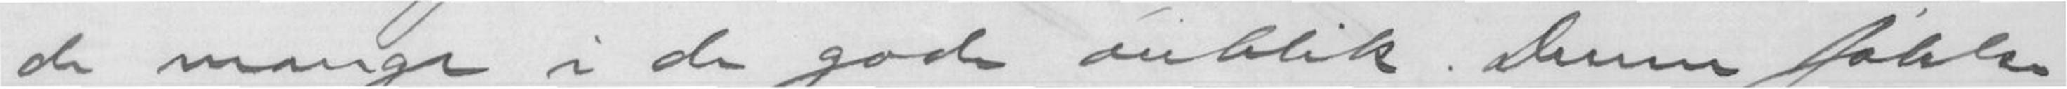de mange i de gode øieblik. Denne følelse
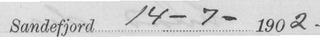Sandefjord 14 - 7 - 1902.
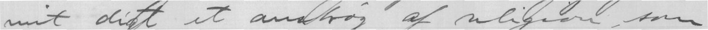mit digt et anstrøg af religiøs, som
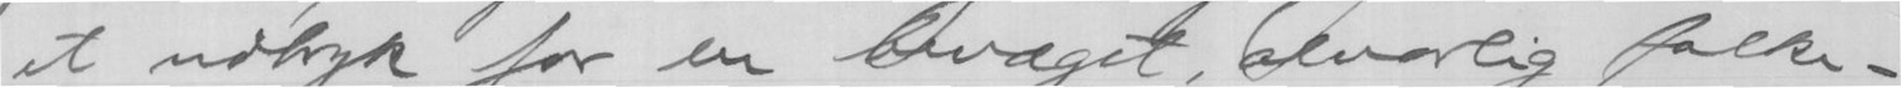et udtryk for en bevæget, alvorlig folke-
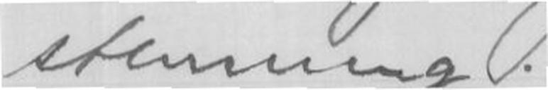stemning.
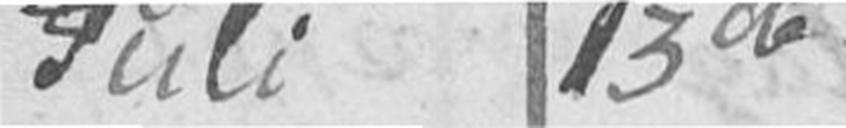Juli 13de
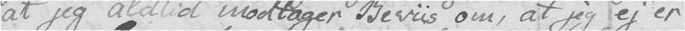at jeg aldtid modtager Beviis om, at jeg ej er
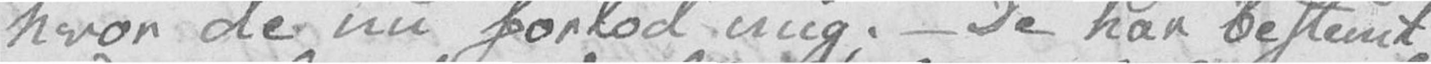hvor de nu forlod mig. – De har bestemt
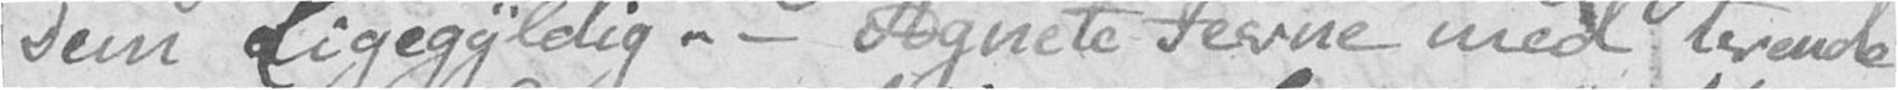Dem Ligegyldig. – Agnete Jevne med tvende
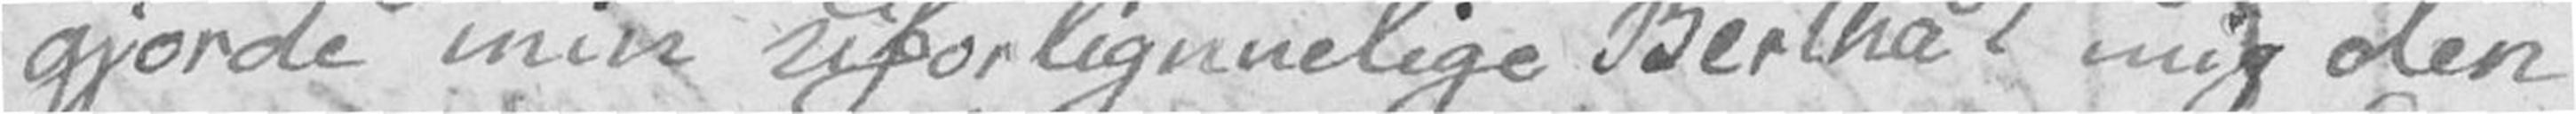gjorde min uforlignnelige Bertha! mig den
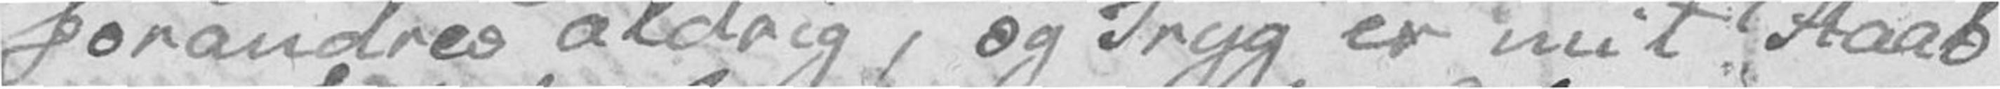forandres aldrig, og Tryg er mit Haab
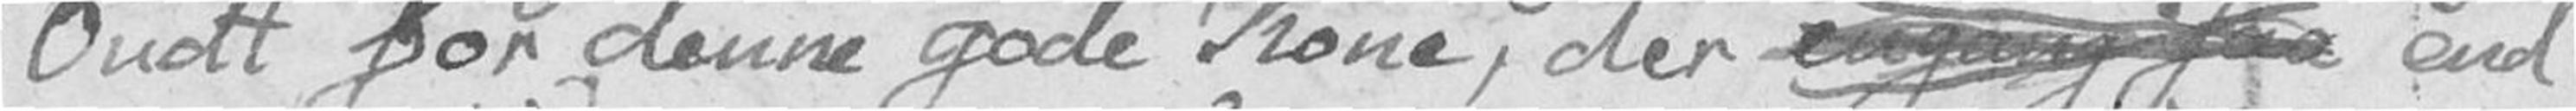Ondt for denne gode Kone, der engang saa end
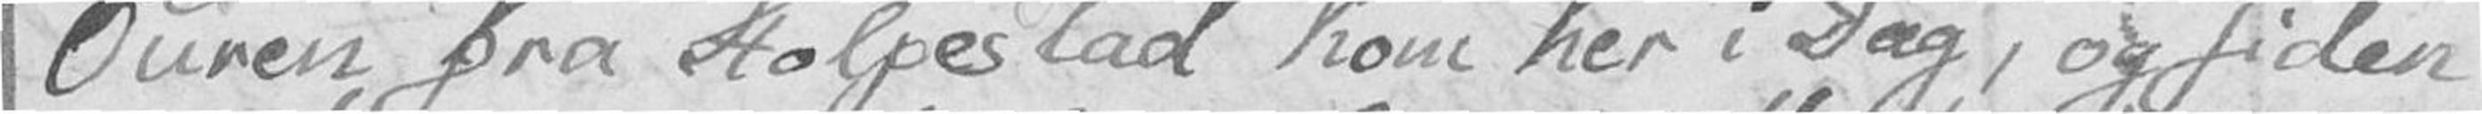Ouren fra Stolpestad kom her i Dag, og siden
